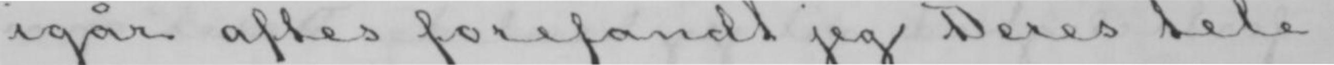igår aftes forefandt jeg Deres tele-
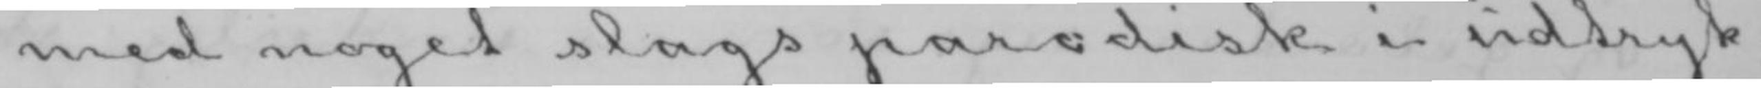med noget slags parodisk i udtryk-
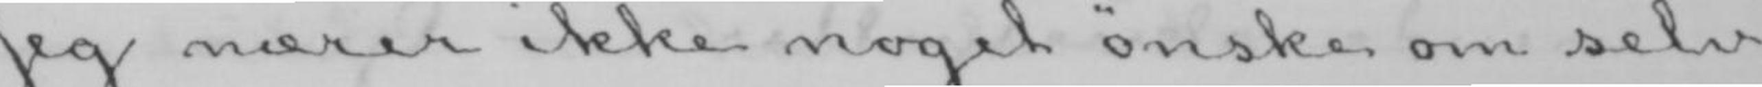Jeg nærer ikke noget ønske om selv
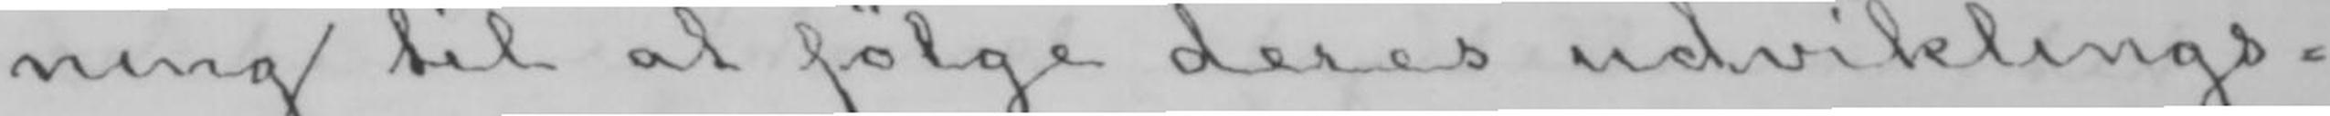ning til at følge deres udviklings-
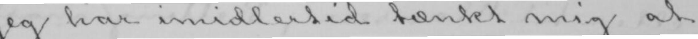Jeg har imidlertid tænkt mig at
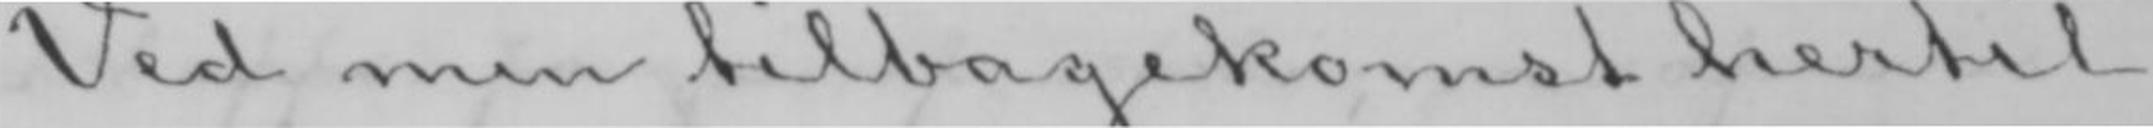Ved min tilbagekomst hertil
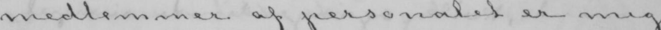medlemmer af personalet er mig
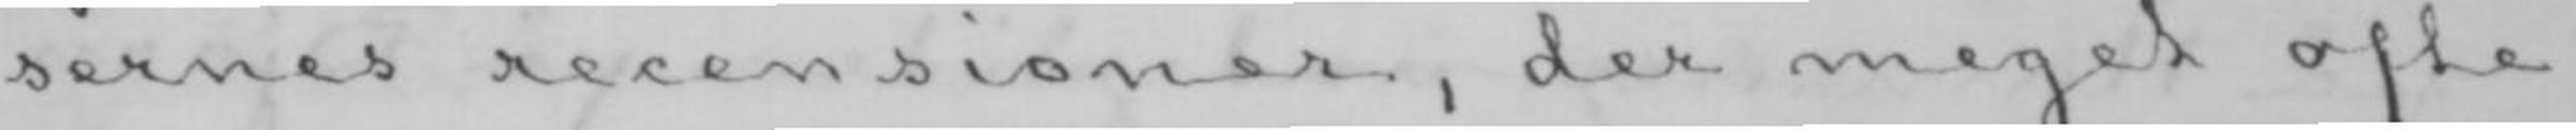sernes recensioner, der meget ofte
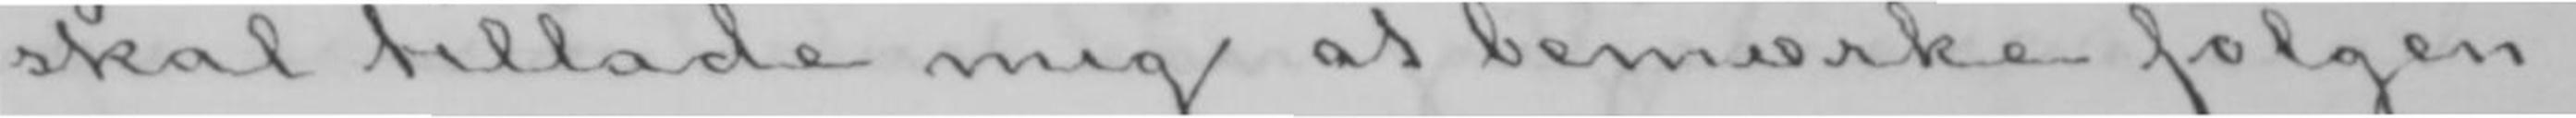skal tillade mig at bemærke følgen-
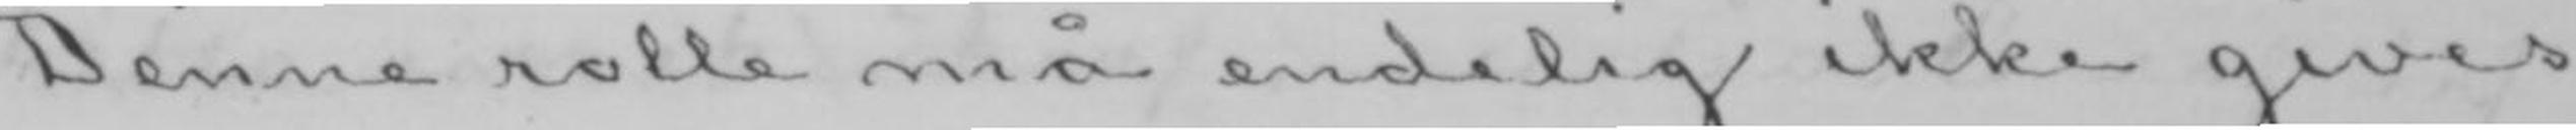Denne rolle må endelig ikke gives
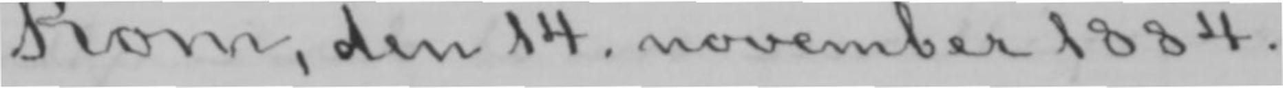Rom, den 14. november 1884.
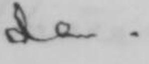de.
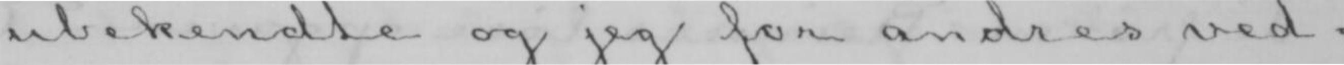ubekendte og jeg for andres ved-
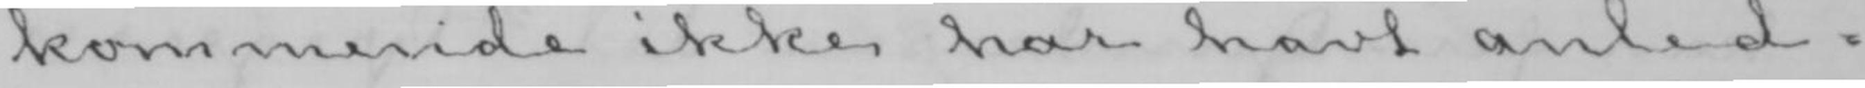kommende ikke har havt anled-
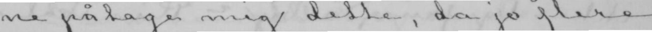ne påtage mig dette, da jo flere
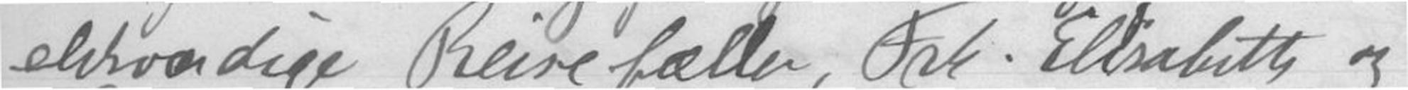elskværdige Reisefæller, Frk. Elisabeth og
